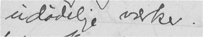udødelige værker.
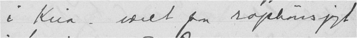i Kria været paa raphønsjagt
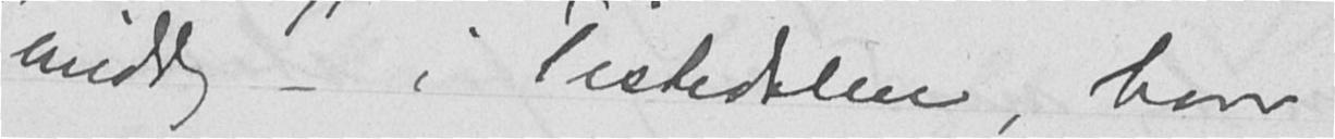middag - i Tistedalen, hvor
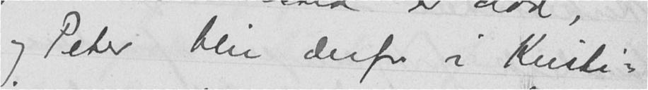og Peter blir derfor i Kristi-
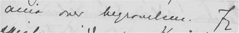ania over begravelsen. Jeg
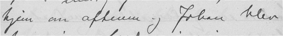hjem om aftenen og Johan blev
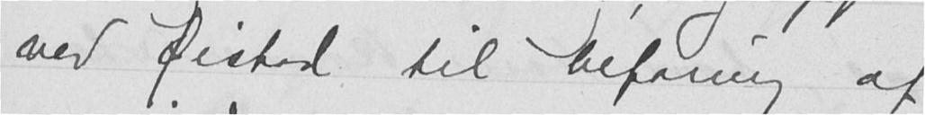ved Øistad til befaring af
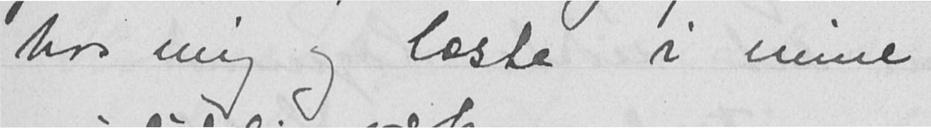hos mig og læste i mine
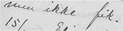men ikke fik.
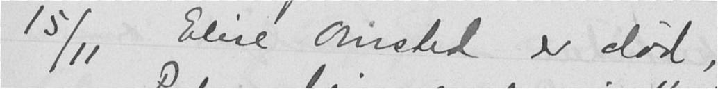15/11 Elise Omsted er død,
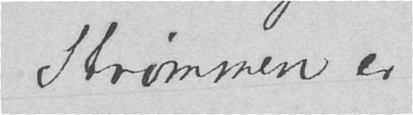Strømmen er
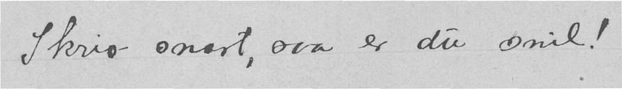Skriv snart, svar er du snil!
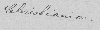Christiania.
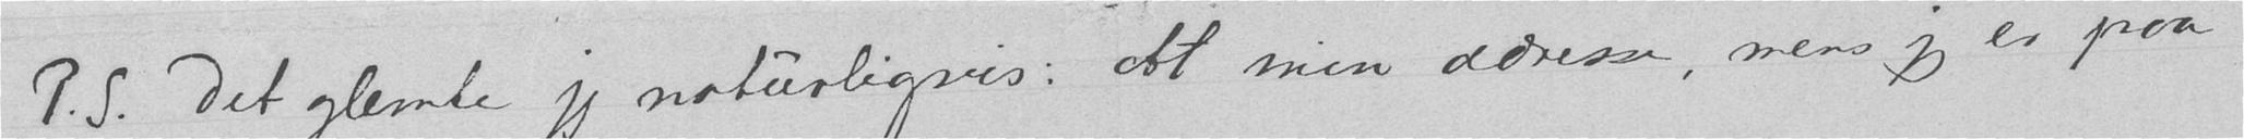P.S. Det glemte jeg naturligvis: At min adresse, mens jeg er paa
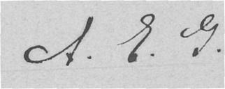A.E.G
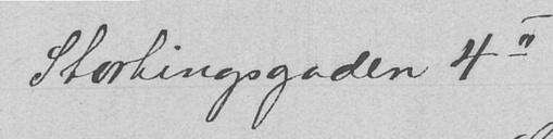Stortingsgaden 4 II
